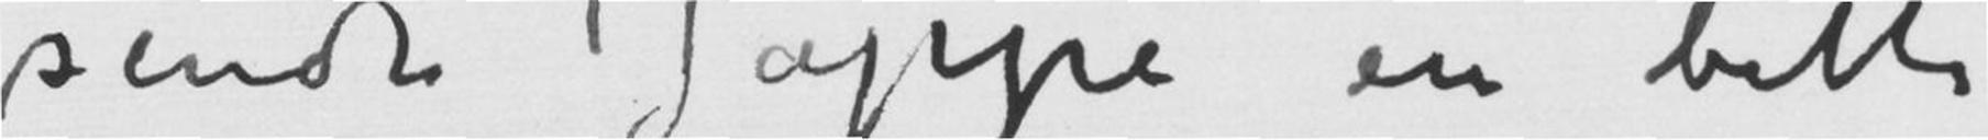sendt Jappe en bitte
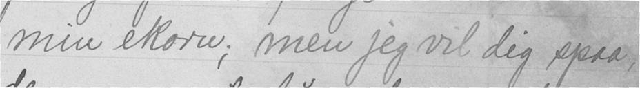min ekorn; men jeg vil dig spaa,
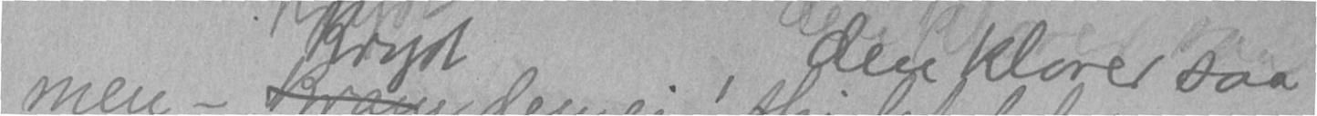men - frykt den ei! den klarer saa
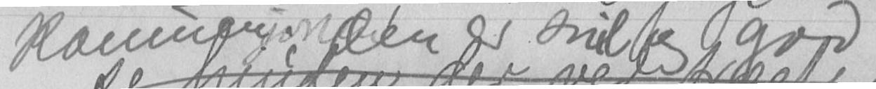kaninen, den er snil og god
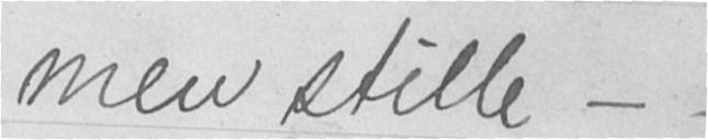men stille -
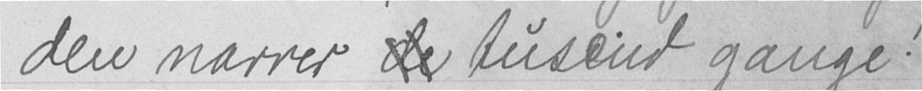den narrer de tusend gange!
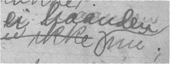ei haanden nu;
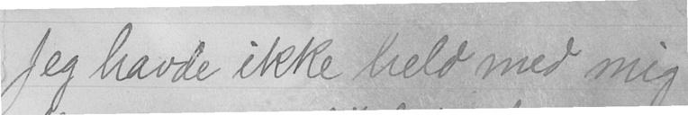Jeg havde ikke held med mig
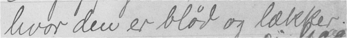hvor den er blød og lækker!
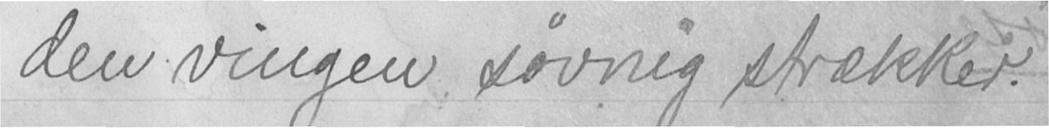den vingen søvnig strækker.
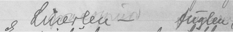Linerlen - fuglen,
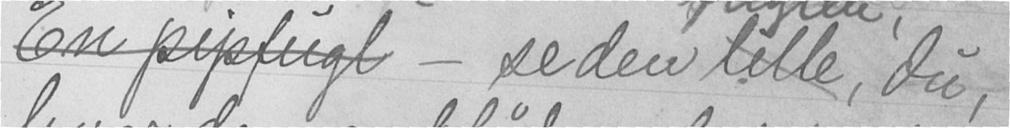En pipfugl - se den lille, du,
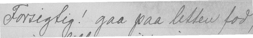Forsigtig! gaa paa letten fod,
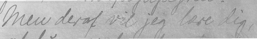Men deraf vil jeg lære dig,
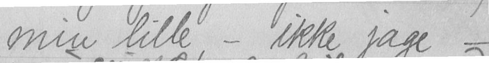min llle - ikke jage -
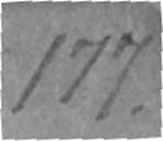177.
